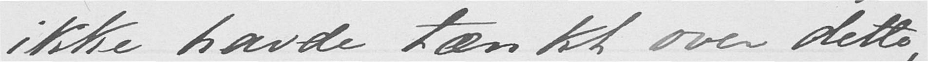ikke havde tænkt over dette,
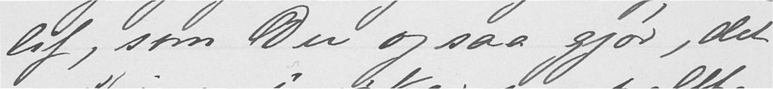lif, som Du ogsaa gjør, det
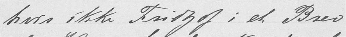hvis ikke Fridtjof i et Brev
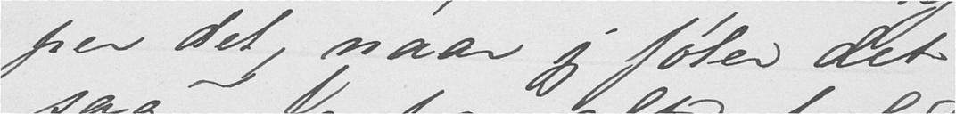per det, naar jeg føler det
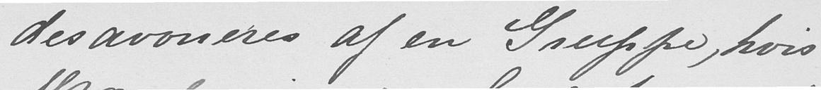desavoueres af en Gruppe, hvis
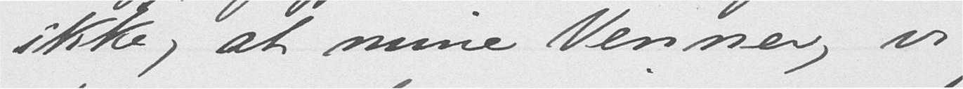ikke, at mine Venner, vi
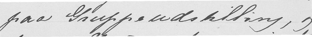paa Gruppeudstilling, og
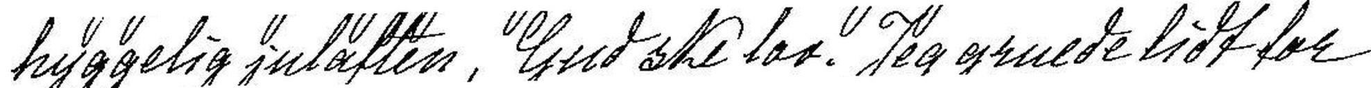hyggelig juleaften, Gud ske lov. Jeg gruede lidt for
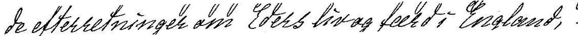de efterretninger om Eders liv og færd i England,
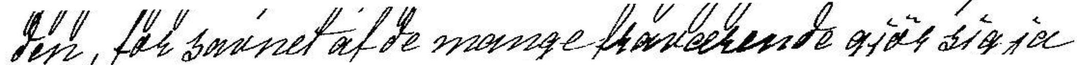den, for savnet af de mange fraværende gjør sig ja
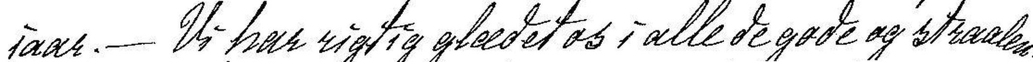iaar. - Vi har rigtig glædet os i alle de gode og straalen-
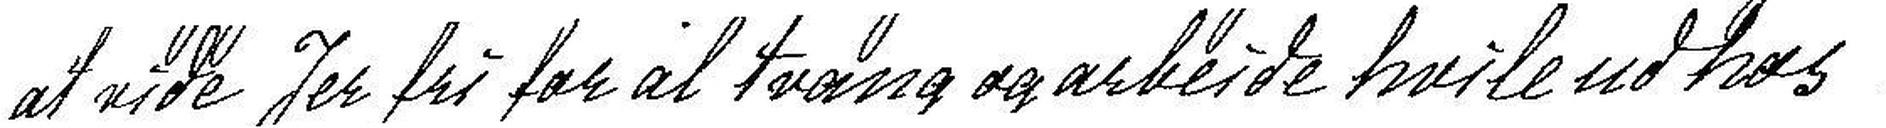at vide Jer fri for al tvang og arbeid hvile ud hos
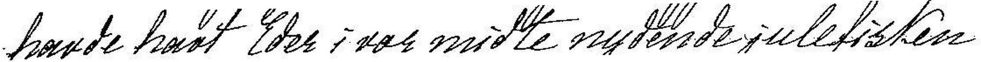havde havt Eder i vor midte nydende julefisken
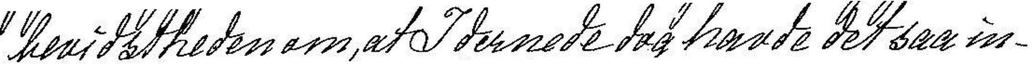bevidstheden om, at I dernede dog havde saa in-
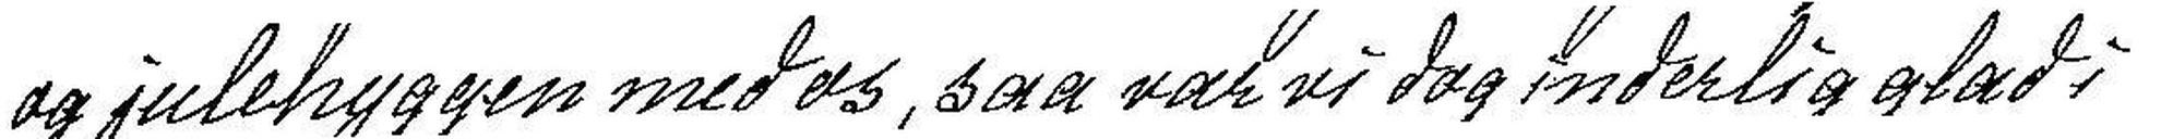og julehyggen med os, saa var vi dog inderlig glad i
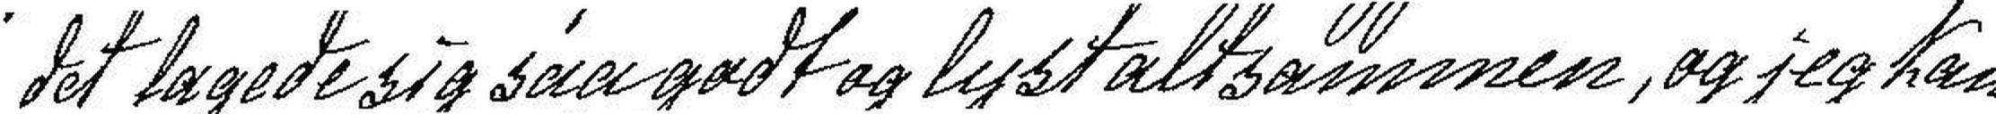det lagede sig saa godt og lyst altsammen, og jeg kan
